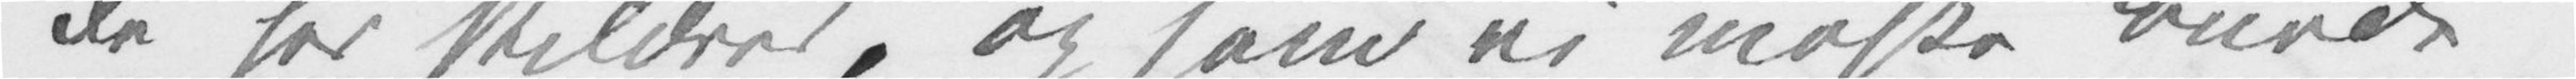De her skildrer, og som vi måske burde
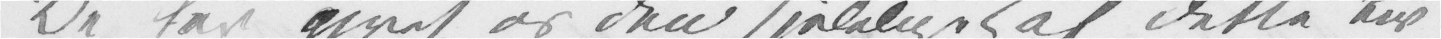De har givet os den sjelelige af dette Liv
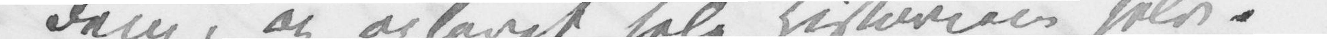dem, og oplevet hele Historien selv.
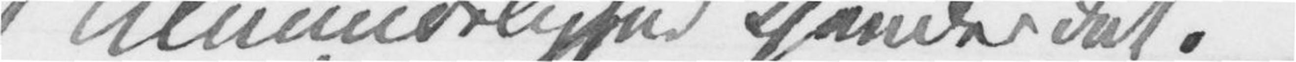i Almindelighed kjender det.
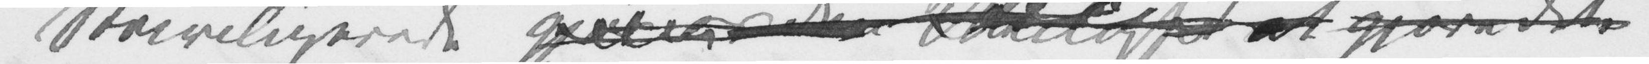«Priviligerede» gjør os den Uleilighed at gjøre det.
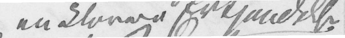en klarere Erkjendelse
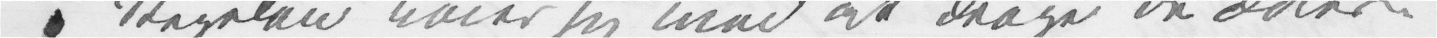i Regelen nøier sig med at drage de ædlere
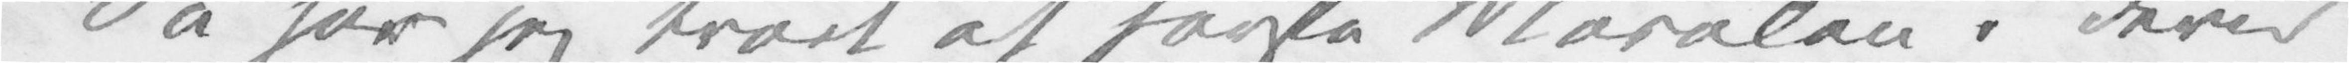Så har jeg troet at forstå Moralen i Deres
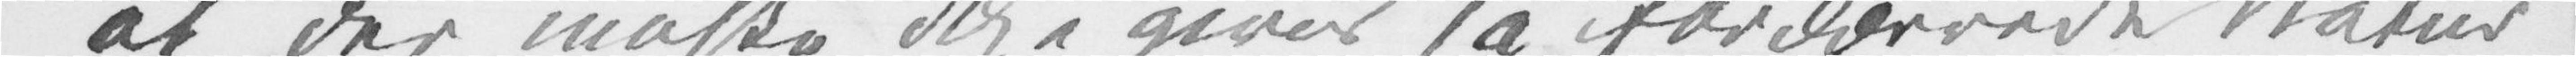at der måske ikke gives så fordærvede Natur¬
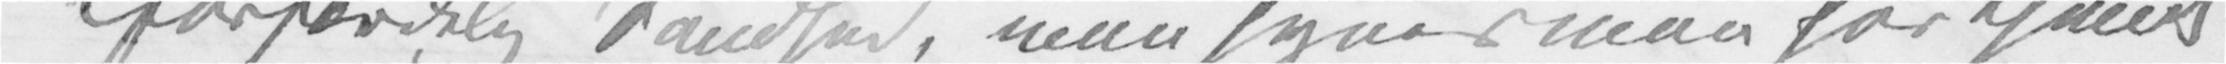forfærdelig Sandhed, man synes man har kjendt
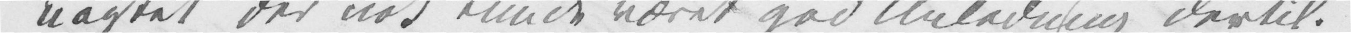uagtet der nok kunde været god Anledning dertil.
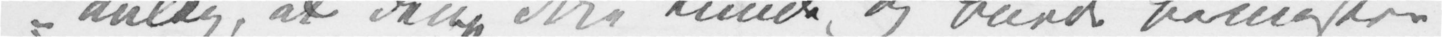anlæg, at denne ikke kunde og burde bemestre
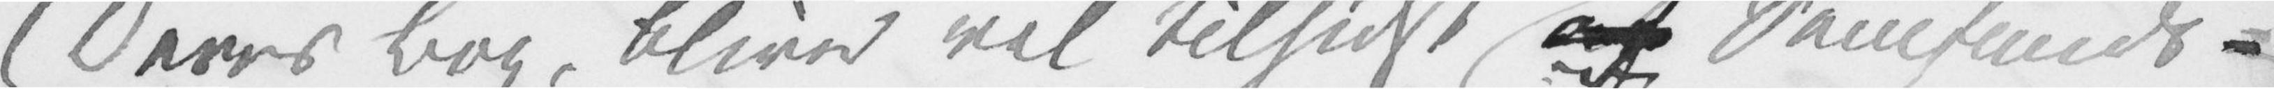Deres Bog, bliver vel tilsidst af Samfunds¬
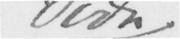Side
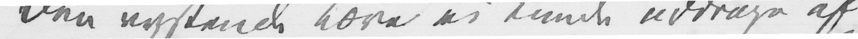Den rystende Lære vi kunde uddrage af
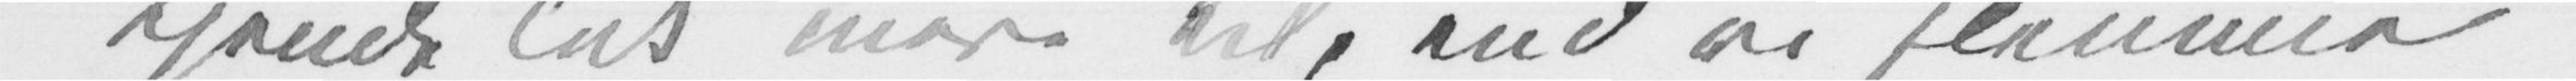kjende lidt mere til, end vi slemme
